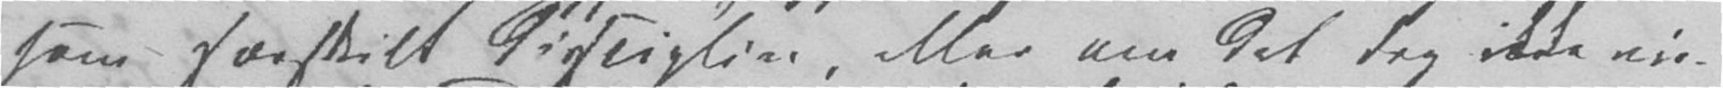som særskilt Disciplin, eller om det dog ikke vir-
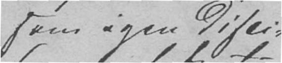som egen Disci-
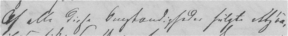Af alle disse Omstændigheder fulgte altsaa,
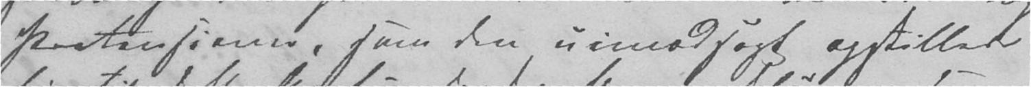Prætensioner, som den uimodsagt opstillede
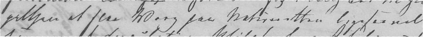pelthen at slaa Vrag paa Naturetten ligesaa vel
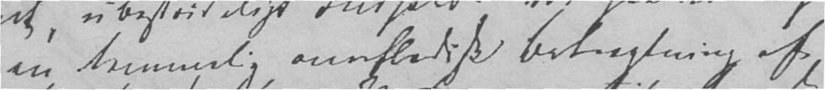en temmelig overfladisk Betragtning af
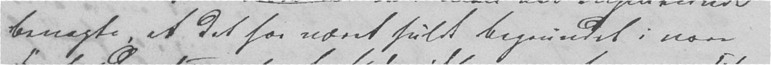benægte, at det har været fuldt begrundet i vore
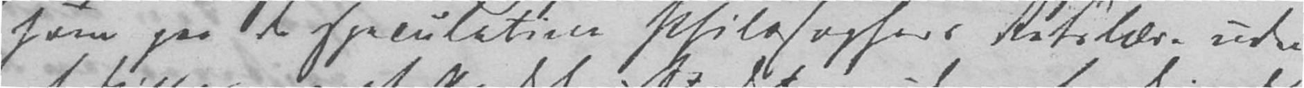som paa de speculative Philosophers Retslære uden
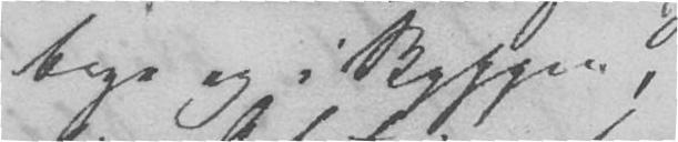bage og i Skyggen,
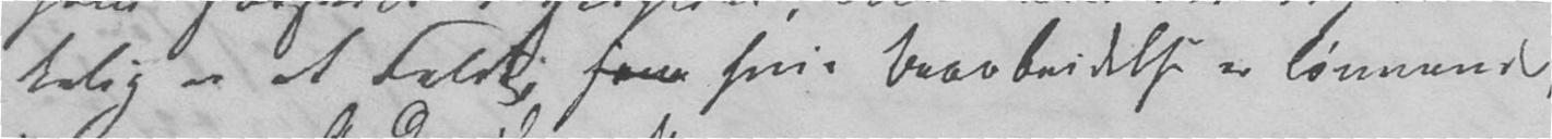kelig er et Feldt, hvis Bearbeidelse er lønnende,
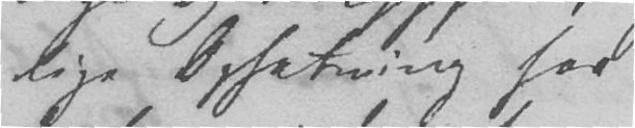lige Opfatning har
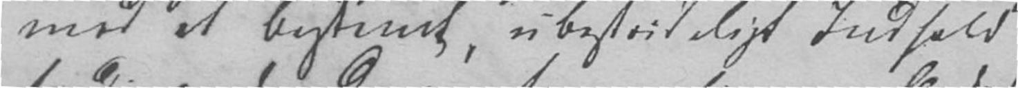med et bestemt, ubestrideligt Indhold
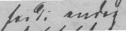fordi endog
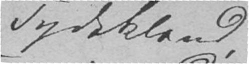Tydskland,
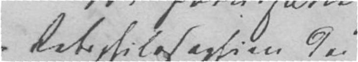Retsphilosophien da
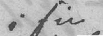i sin
